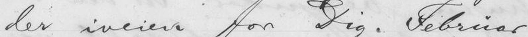der iveien for Dig. Februar
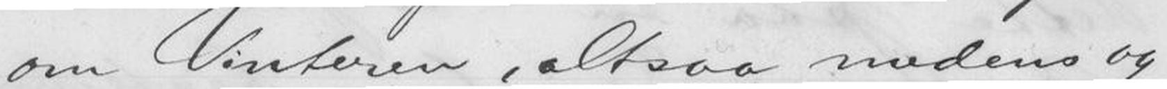om Vinteren, altsaa medens og-
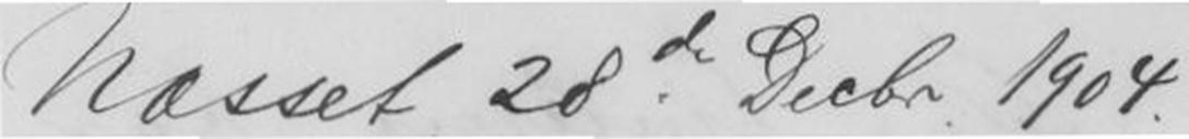Næsset, 28de Decbr. 1904.
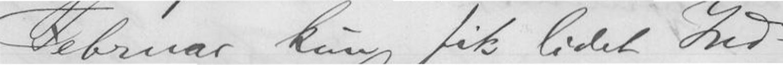Februar kun fik lidet Ind-
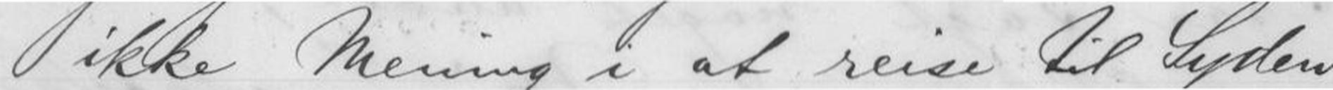ikke Mening i at reise til Syden
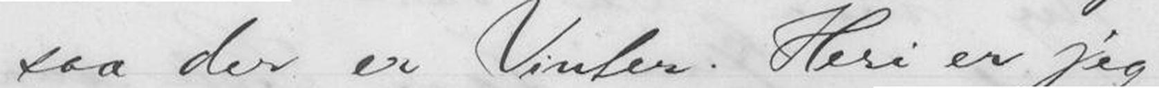saa der er Vinter. Heri er jeg
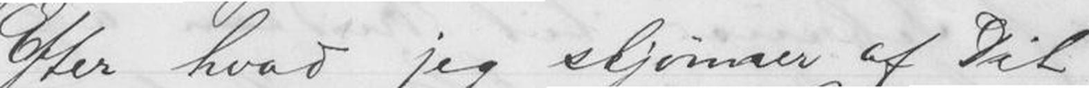Efter hvad jeg skjønner af Dit
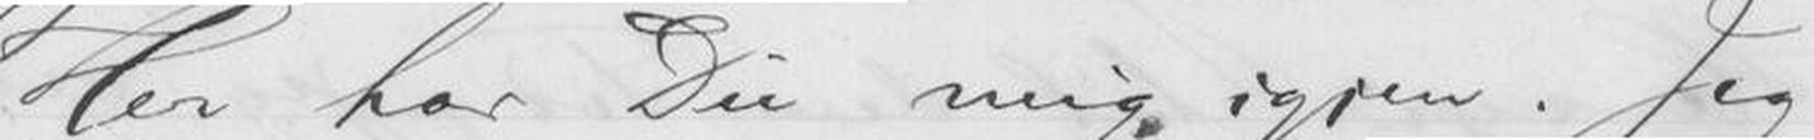Her har du mig igjen. Jeg
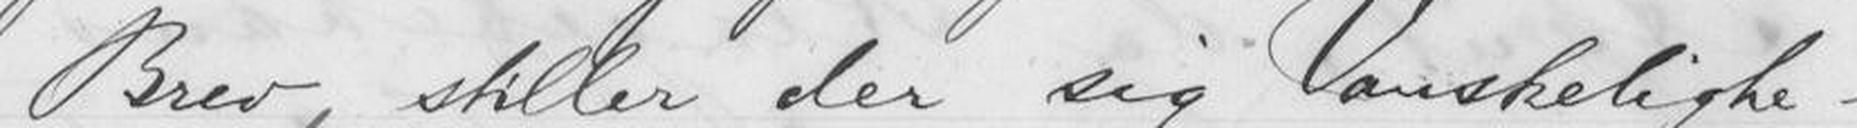Brev, stiller der sig Vanskelighe-
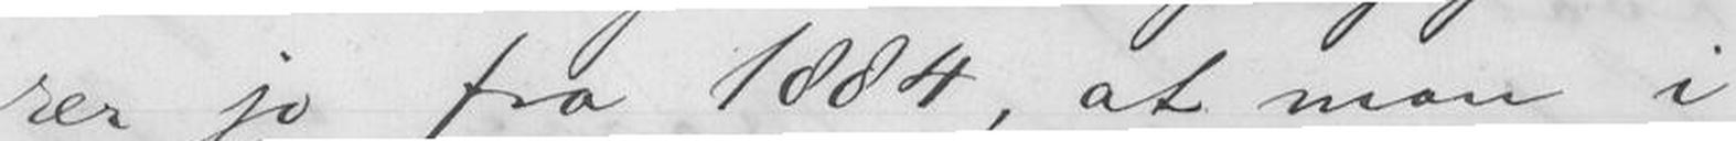rer jo fra 1884, at man i
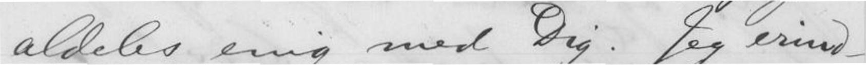aldeles enig med Dig. Jeg erind-
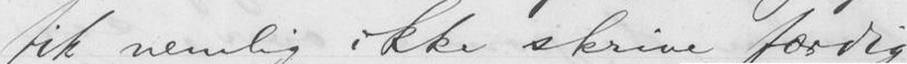fik nemlig ikke skrive færdig
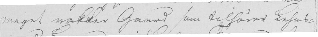meget vakker Gaard som tilhører Lehns-
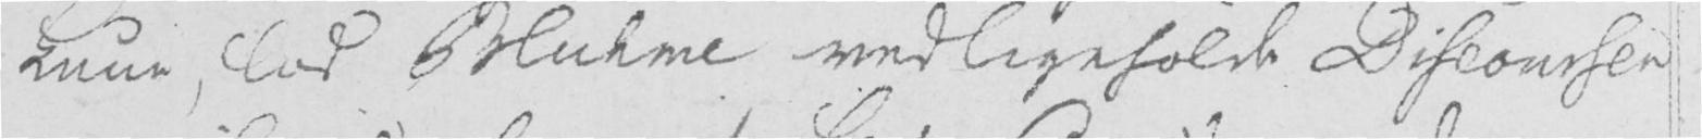Lune, lod Bluhme vedligeholde Discoursen
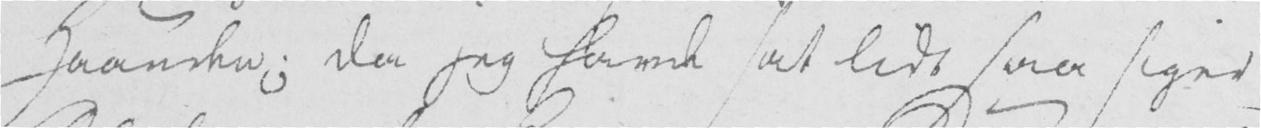Haanden; da jeg havde sat lidt saa siger
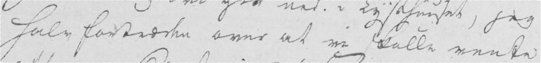halv fortræden over at vi skulle vente
